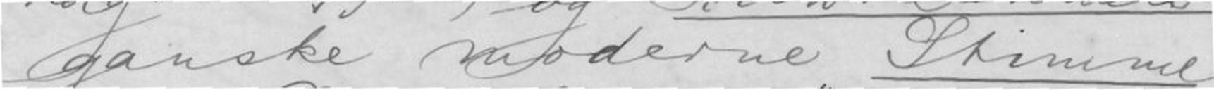ganske moderne "Stimme
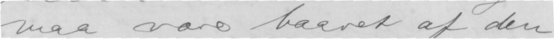maa være baaret af den
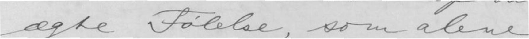ægte Følelse, som alene
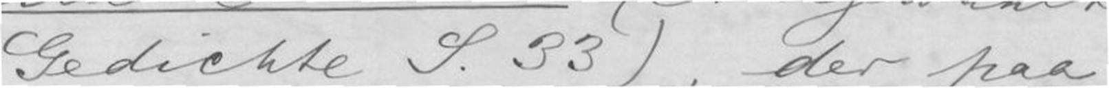Gedichte S.33 ), der paa
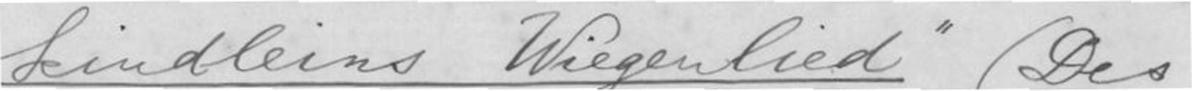Kindleins Wiegenlied (Des
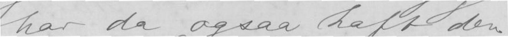har da ogsaa haft den
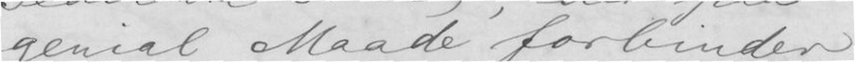genial Maade forbinder
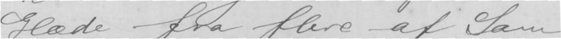Glæde fra flere af Sam
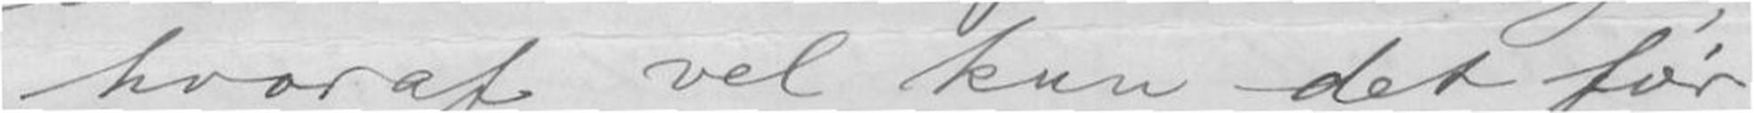hvoraf vel kun det før
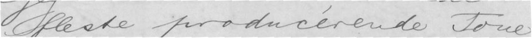fleste producerende Tone
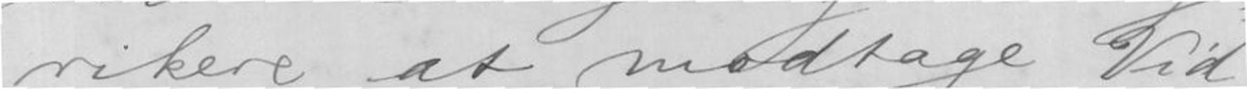rikere at modtage Vid-
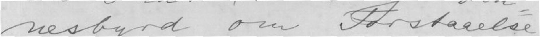nesbyrd om Forstaaelse
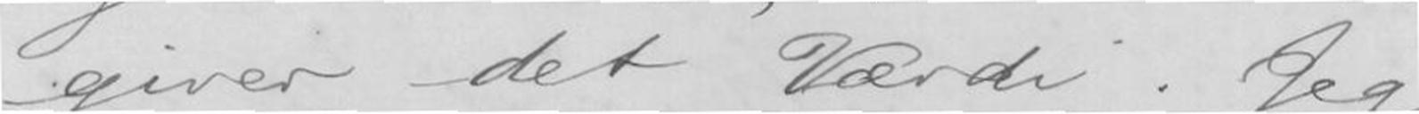giver det Værdi. Jeg
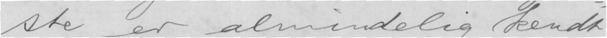ste er almindelig kendt,
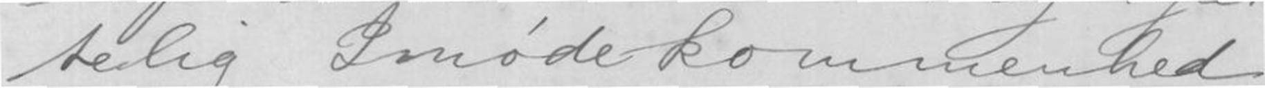telig Imødekommenhed
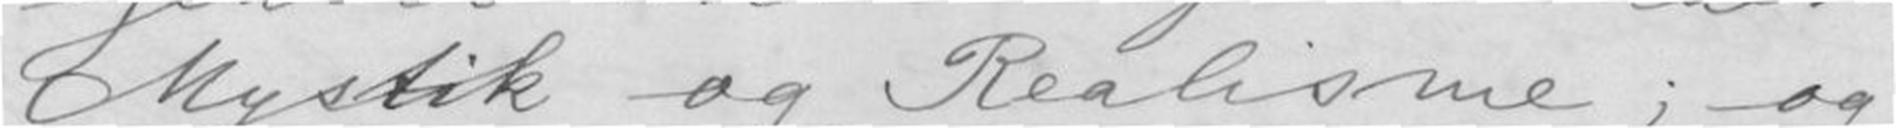Mystik og Realisme; og
